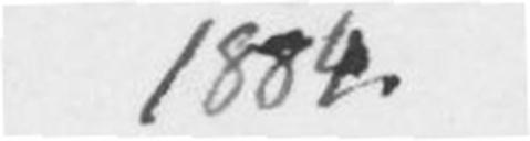1884.
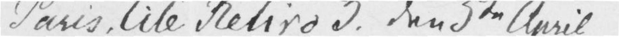Paris, Cité Retiro 3. Den 5te April
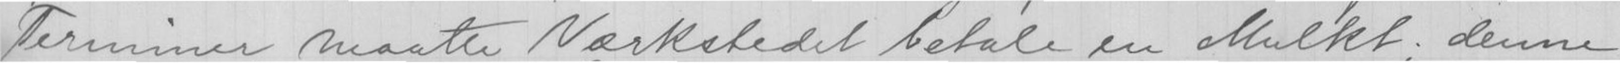Terminer maatte Værkstedet betale en Mulkt; denne
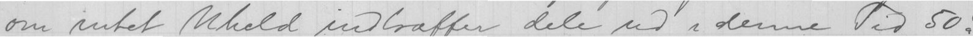om intet Uheld intræffer dele ud i denne Tid 50 à
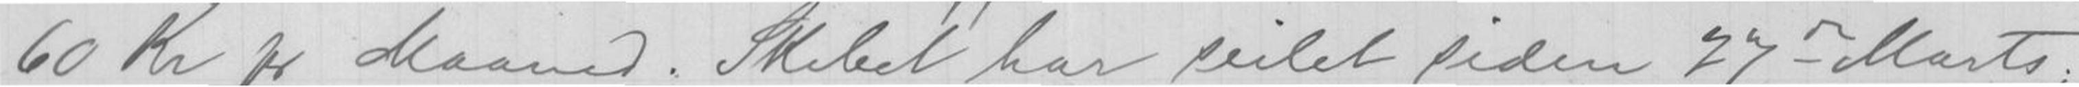60 Kr pr Maaned. Skibet har seilet siden 27de Marts;
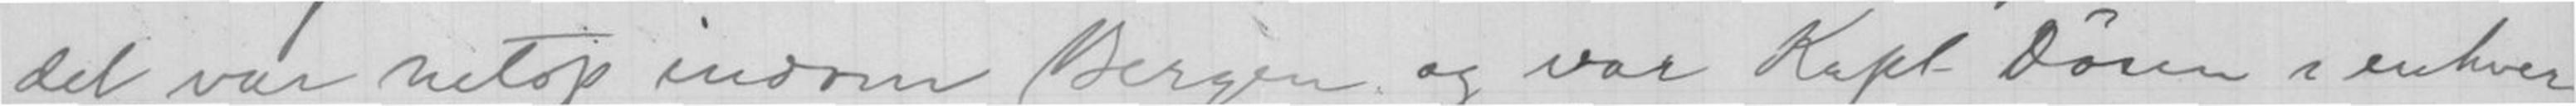det var netop indom Bergen og var Kapt Døsen i enhver
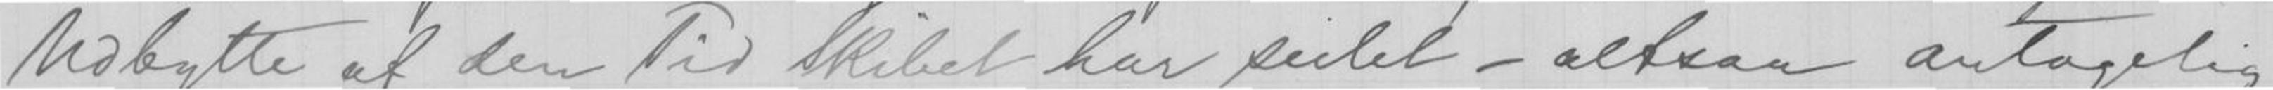Udbytte af den Tid Skibet har seilet - altsaa antagelig
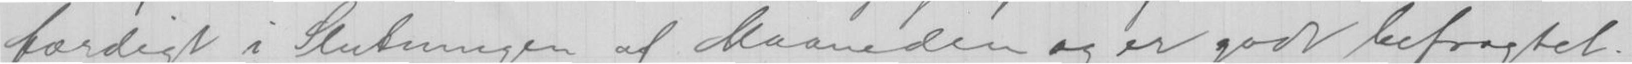færdigt i Slutningen af Maaneden og er godt befragtet.
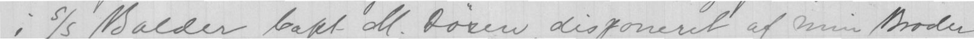i s/s Balder, Capt M Døsen, disponeret af min Broder
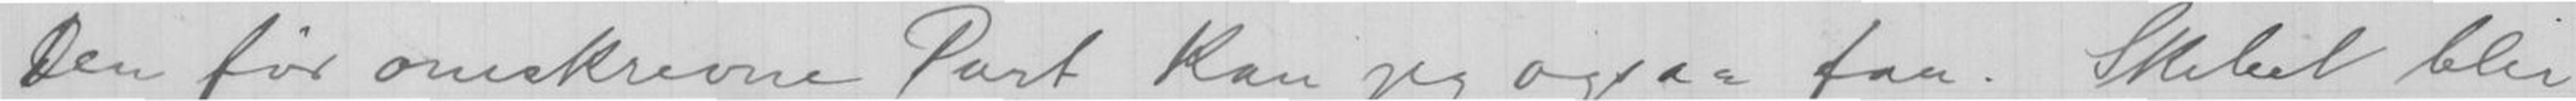Den før omskrevne Part kan jeg ogsaa faa. Skibet blir
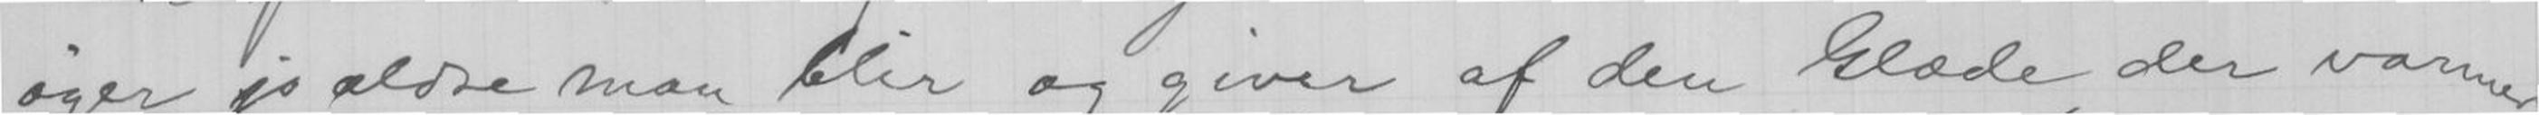øger jo ældre man blir og giver af den Glæde der varmer
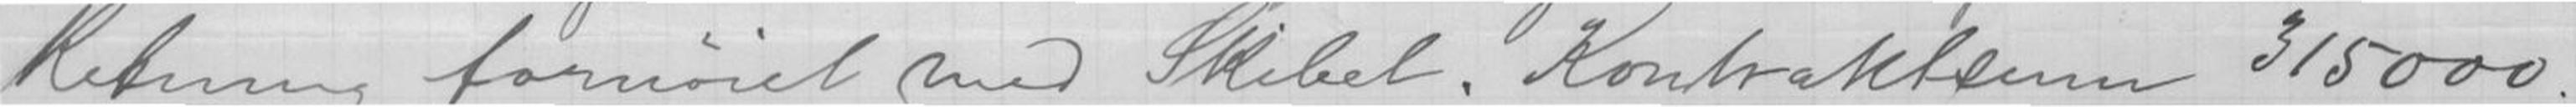Retning fornøiet med Skibet. Kontraktsum 315,000;
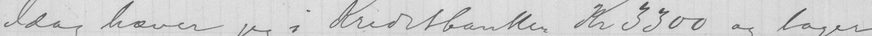Idag hæver jeg i Kreditbanken Kr 3300. og tager
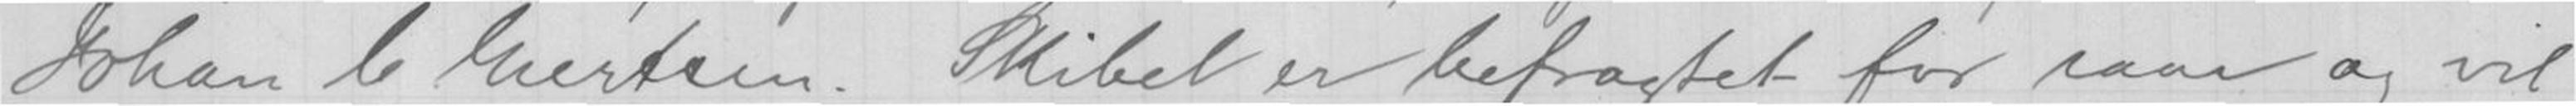Johan C Giertsen. Skibet er befragtet for iaar og vil
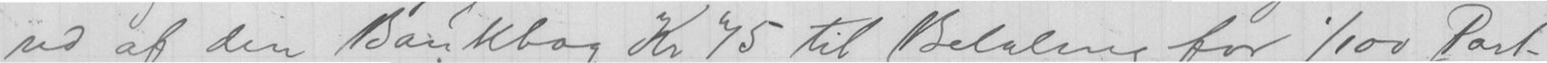ud af din Bankbog Kr 75 til Betaling for 1/100 Part
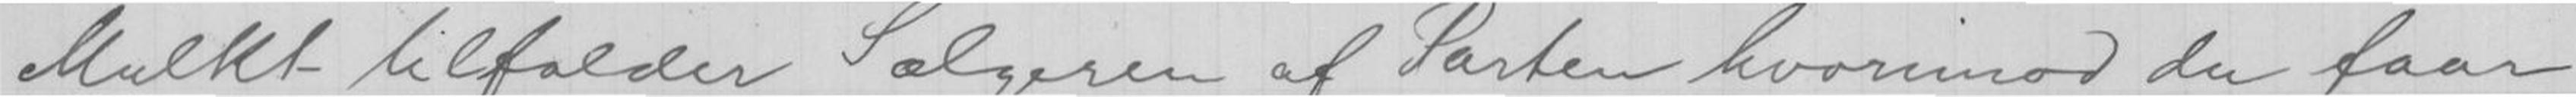Mulkt tilfalder Sælgeren af Parten hvorimod du faar
